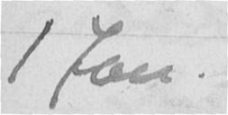1 Jan.
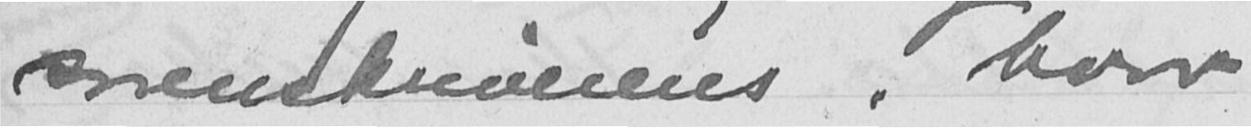sorenskriverens, hvor
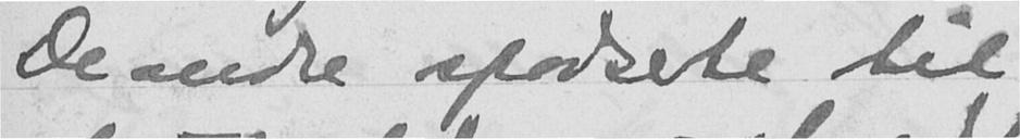De andre spadserte til
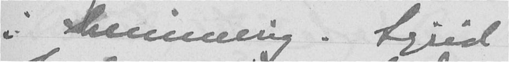i himmerig. Sigrid
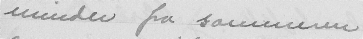minder fra sommeren
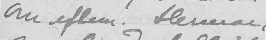Om efterm. Herman,
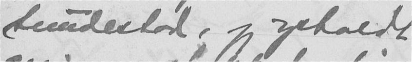Lundestad, jeg opholdt
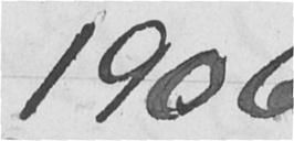1906
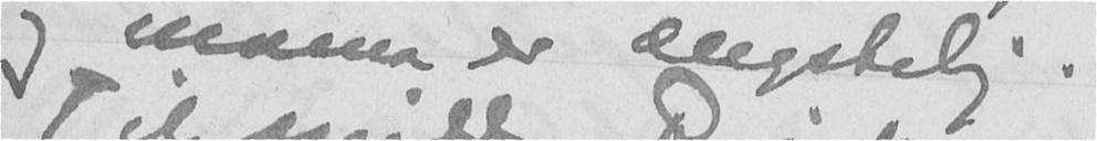og mama er ængstelig.
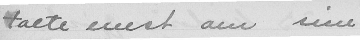talte mest om sine
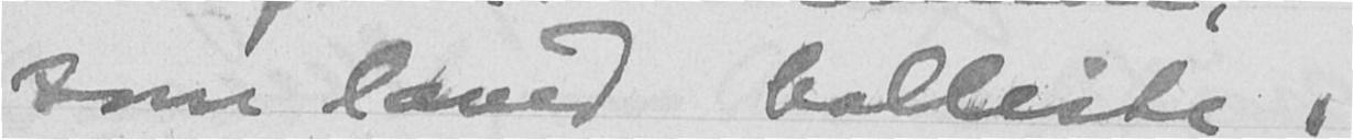som laved balliste.
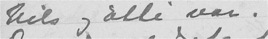Nils og Etti var.
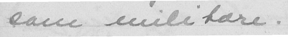som militære.
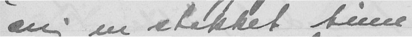mig en stakket time
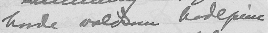havde voldsom hodepine
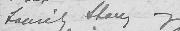Lauritz Stang og
